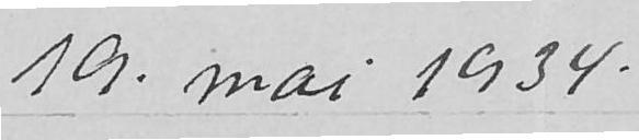19. mai 1934.
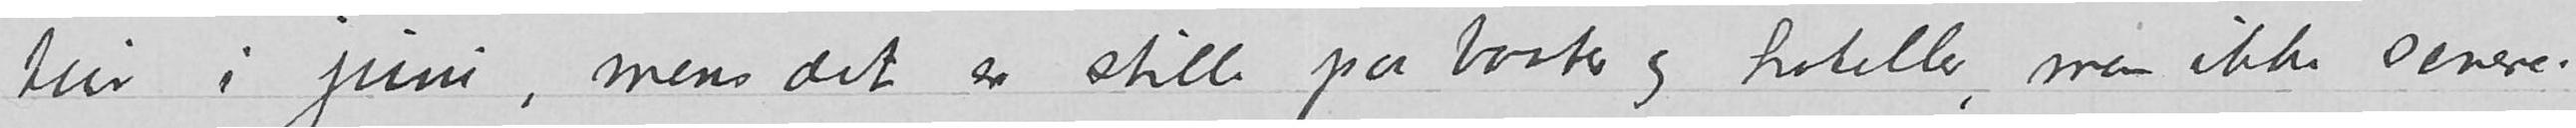tur i juni, mens det er stille paa baater og hoteller, men ikke senere.
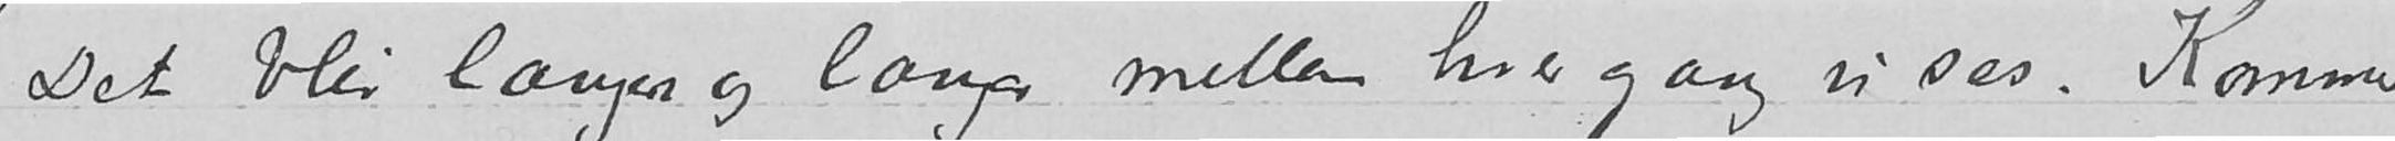Det blir længer og længer mellem hver gang vi ses. Kommer
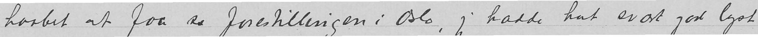haabet at faa se forestillingen i Oslo, jeg hadde hat svært god lyst
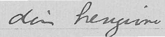din hengivne
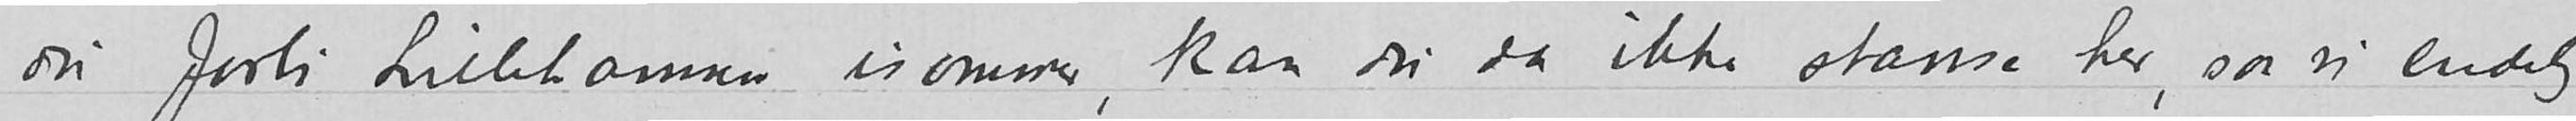du forbi Lillehammer isommer, kan du da ikke stanse her, saa vi endelig
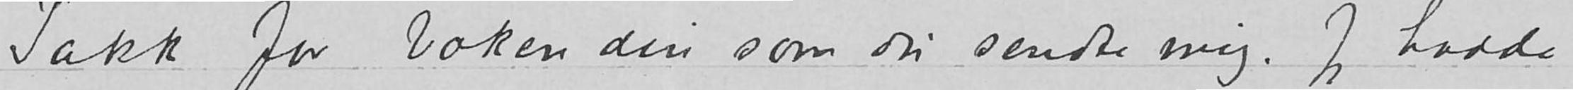Takk for boken din som du sendte mig. Jeg hadde
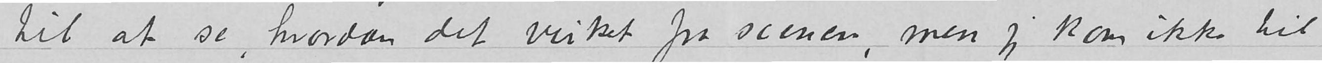til at se, hvordan det virket fra scenen, men jeg kom ikke til
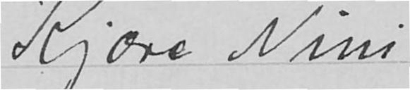Kjære Nini,
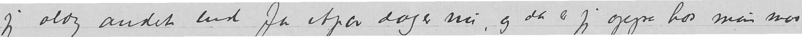jeg aldrig andet end for etpar dager nu, og da er jeg oppe hos min mor
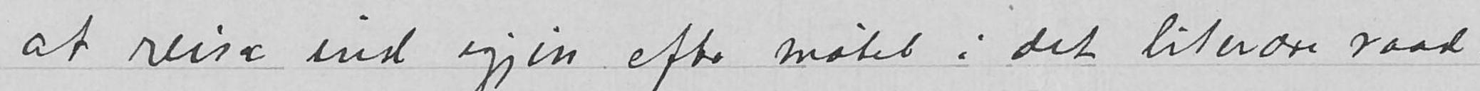at reise ind igjen efter møtet i det literære raad.
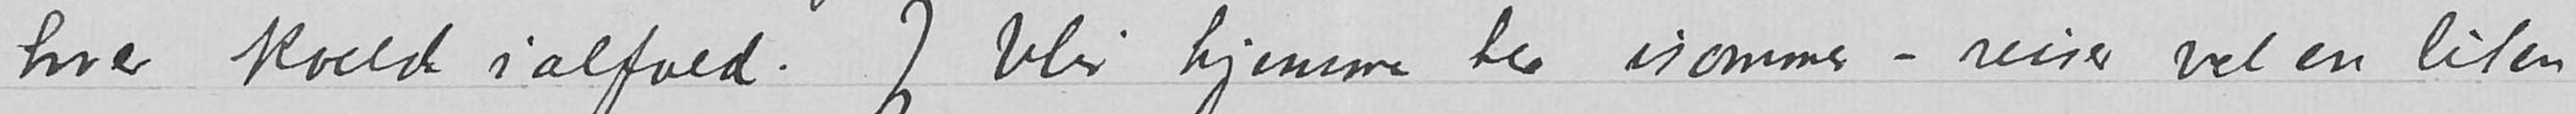hver kveld ialfald. Jeg blir hjemme her isommer – reiser vel en liten
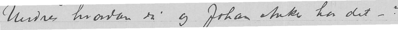Undres hvordan du og Johan Anker har det –?
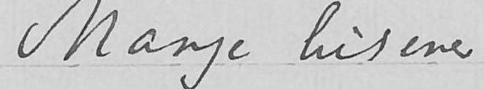Mange hilsener
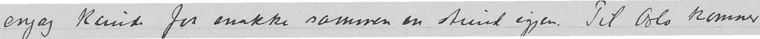engang kunde faa snakke sammen en stund igjen. Til Oslo kommer
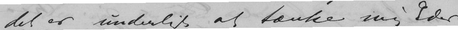det er underligt at tænke mig Eder
